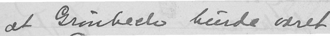at Grønbech burde været
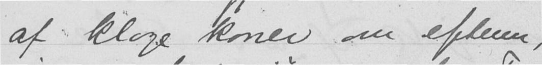af kloge koner om efterm,
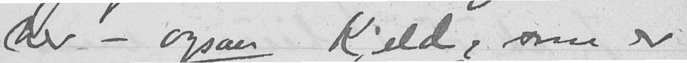her - ogsaa Kjeld, som er
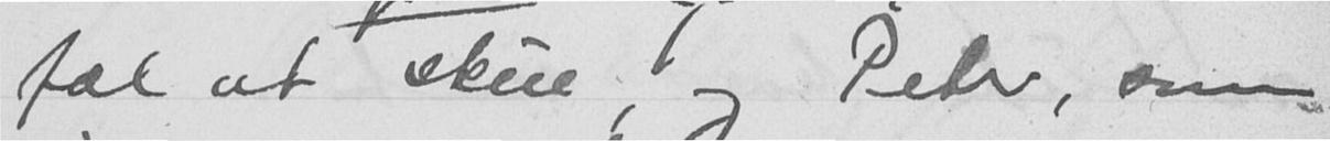fæl at skue, og Peter, som
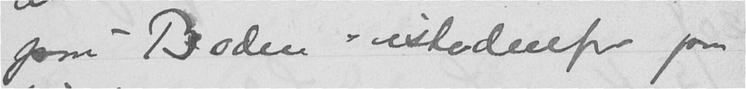paa "Boden" istedenfor paa
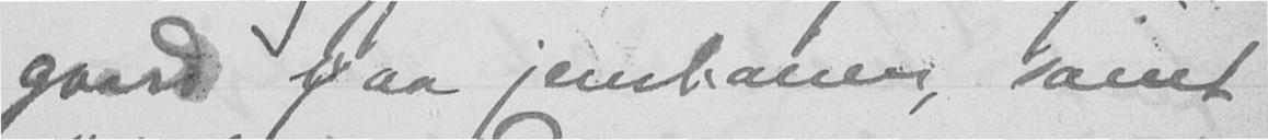gaard paa jenbanen, samt
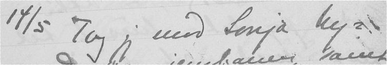14/5 Tog jeg mod Sonja Ny?
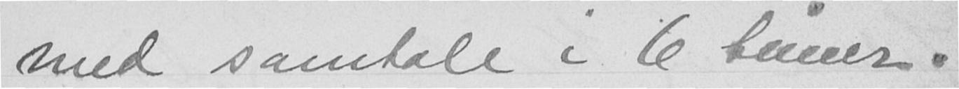med samtale i 6 timer.
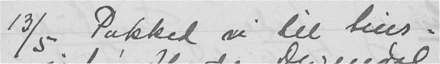13/5 Pakked vi til tiur-
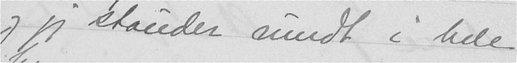og jeg stauder rundt i hele
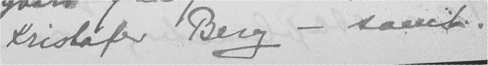Kristofer Berg - samt
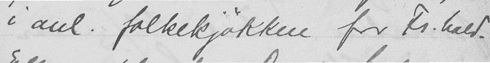i anl. folkekjøkken for Fr. hald.
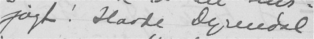jagt! Havde Dyrendal
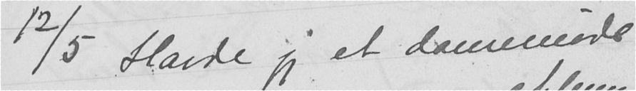12/5 Havde jeg et damemøde
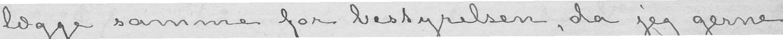lægge samme for bestyrelsen, da jeg gerne
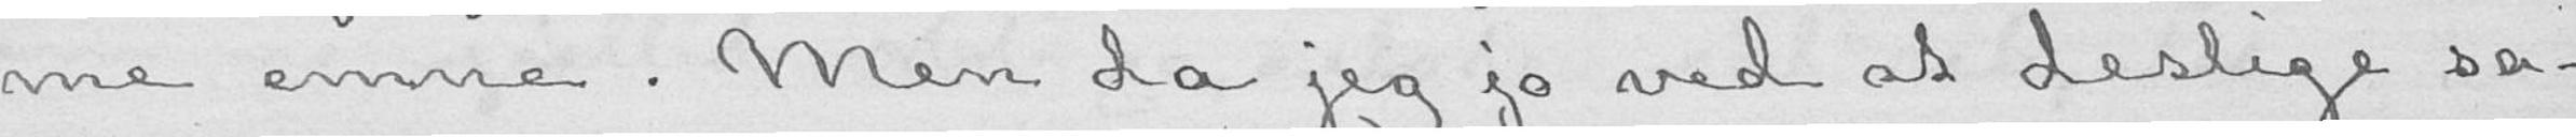me emne. Men da jeg jo ved at deslige sa-
